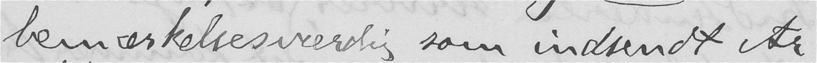bemærkelsesværdig som indsendt Ar-
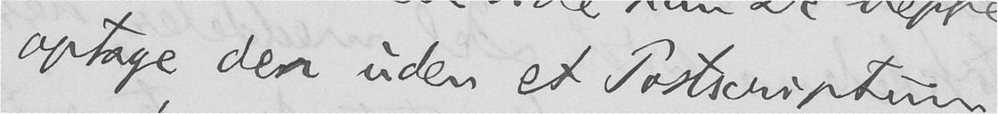optage den uden et Postscriptum,
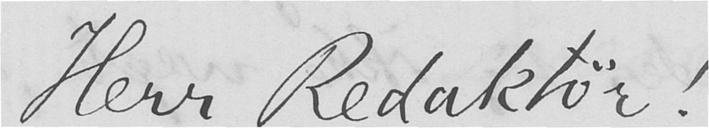Herr Redaktør!
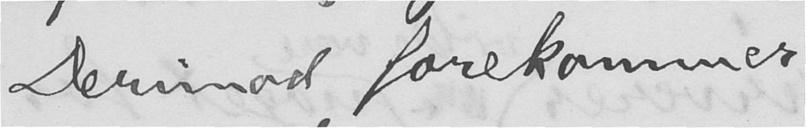Derimod forekommer
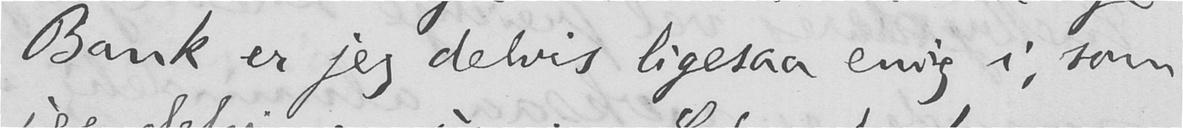Bank er jeg delvis ligesaa enig i, som
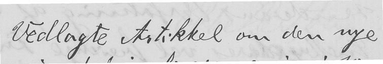Vedlagte Artikkel om den nye
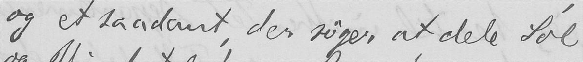og et saadant, der søger at dele Sol
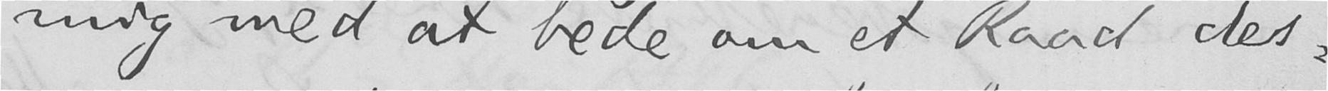mig med at bede om et Raad des-
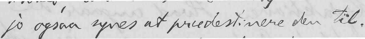jo ogsaa synes at prædestinere den til.
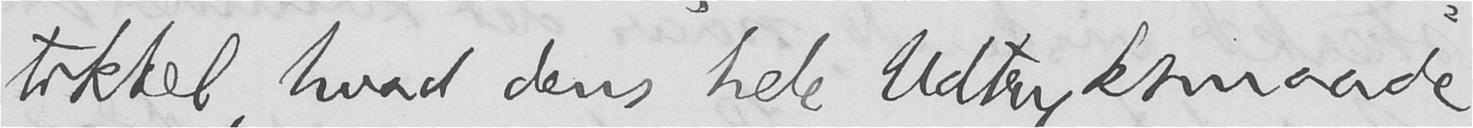tikkel, hvad dens hele Udtryksmaade
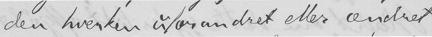den hverken uforandret eller ændret
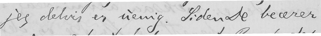jeg delvis er uenig. Siden De beærer
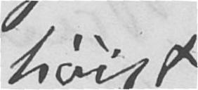høist
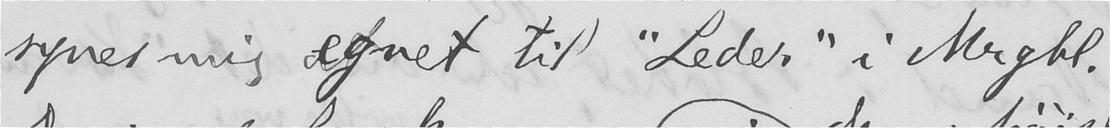synes mig egnet til "Leder" i Mrgbl.
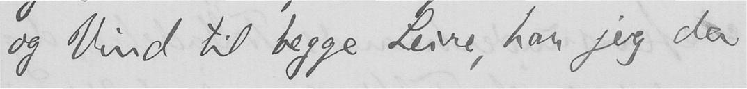og Vind til begge Leire, har jeg da
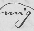mig
